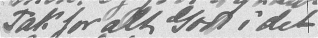Tak for alt Godt i det
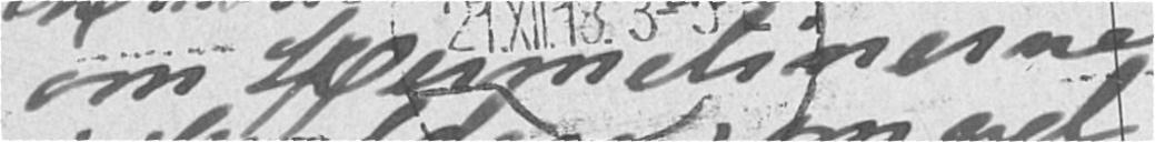om Hermelinerne
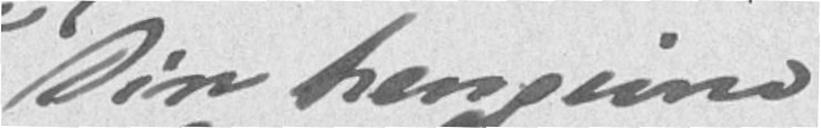Din hengivne
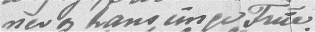ner og hans unge Frue.
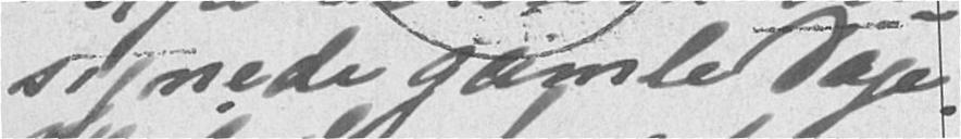signede gamledage.
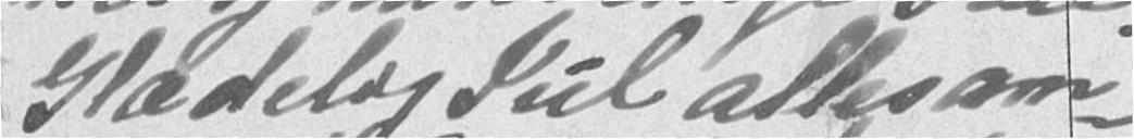Glædelig Jul allesam-
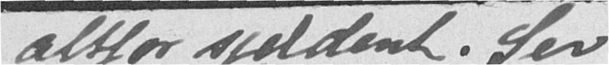altfor sjeldent. Ser
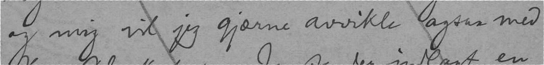og mig vil jeg gjærne avvikle ogsaa med
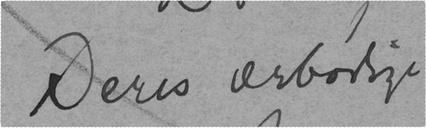Deres ærbødige
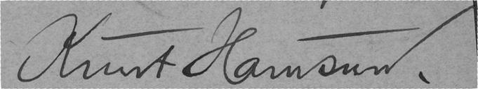Knut Hamsun.
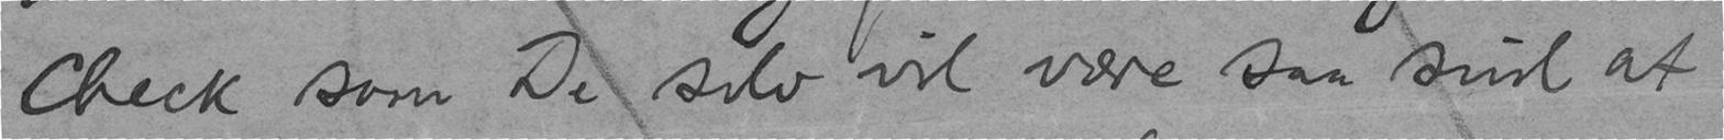Check som De selv vil være saa snil at
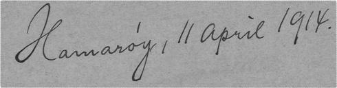Hamarøy, 11 April 1914.
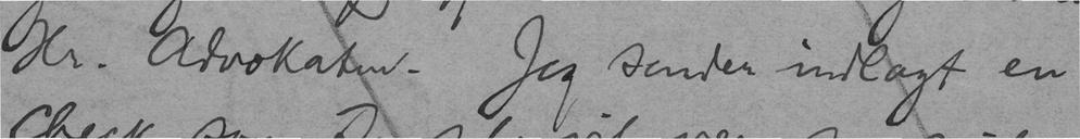Hr. Advokaten. Jeg sender indlagt en
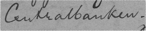Centralbanken.
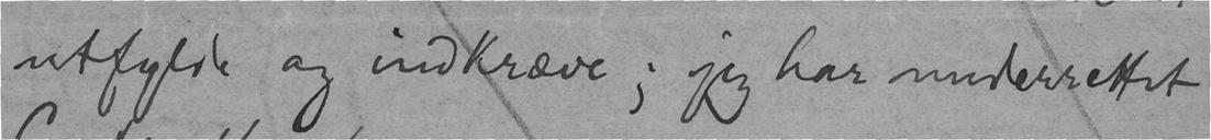utfylde og indkræve; jeg har underrettet
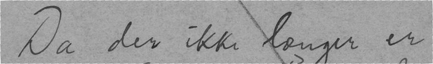Da der ikke længer er
